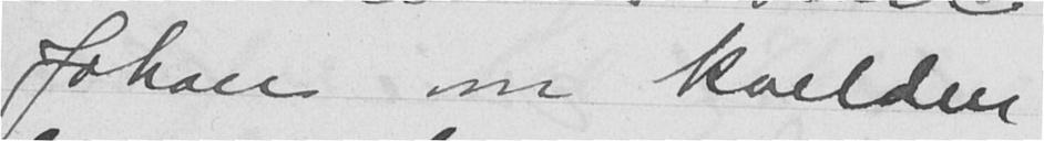Johan om kvelden,
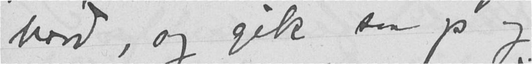bord, og gik saa op og
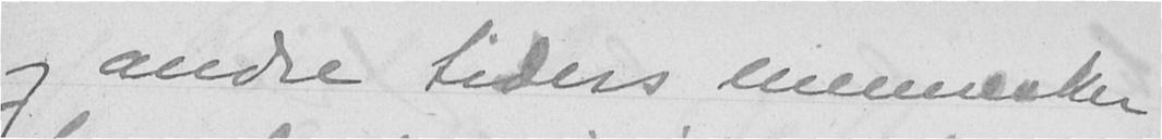og andre tiders mennesker
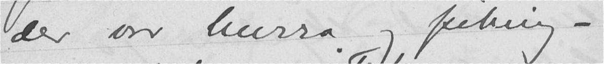der var hurra og pibing -
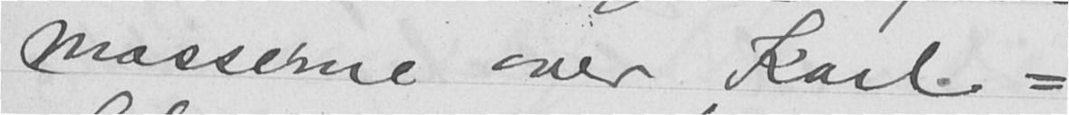masserne over Karl-
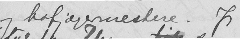og hofjægermestere. Jeg
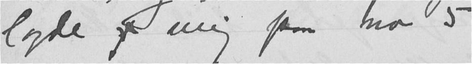lagde p mig paa no 5
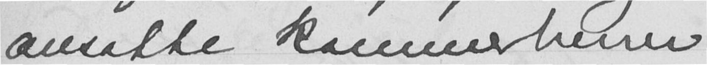ansatte kammerherrer
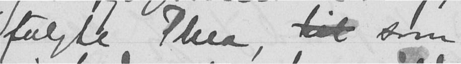fulgte Thea, til som
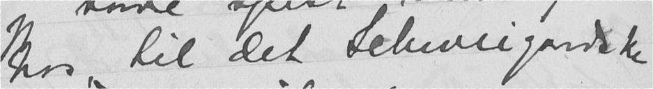hos, til det Schweigaardske
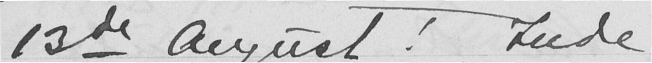13de august! Inde
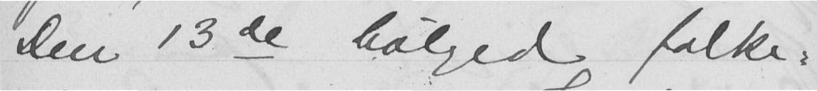Den 13de bølged folke-
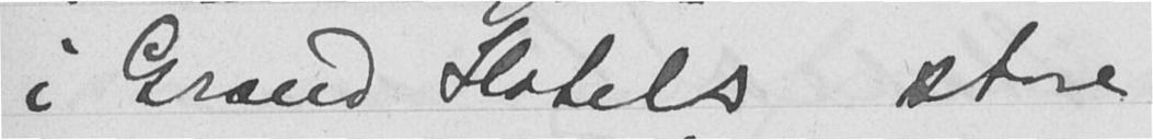i Grand Hotels store
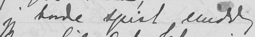jeg havde spist middag
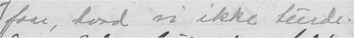faar, hvad vi ikke turde.
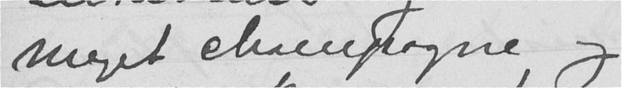meget champagne og
